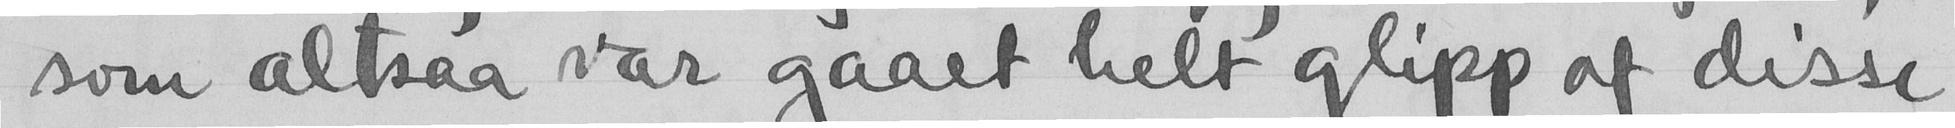som altsaa var gaaet helt glipp af disse
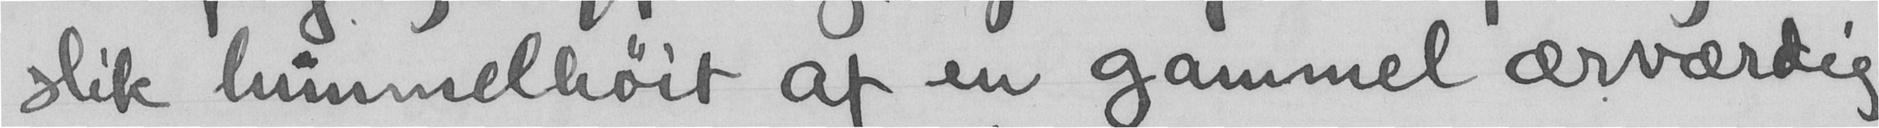slik hunmelhøit af en gammel ærværdig
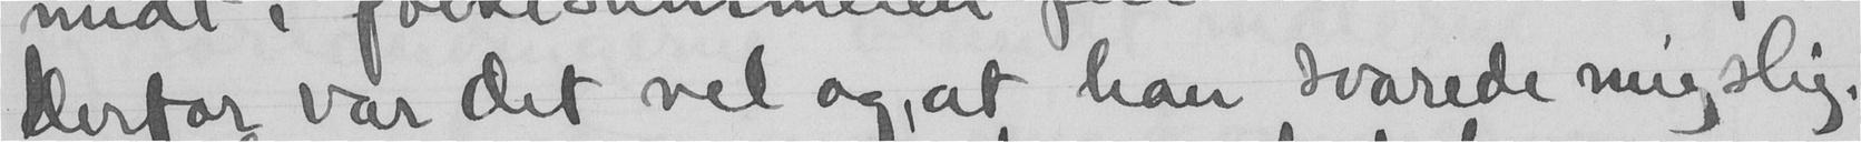derfor var det vel og, at han svarede mig slig,
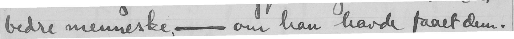bedre menneske, - om han havde faaet dem.
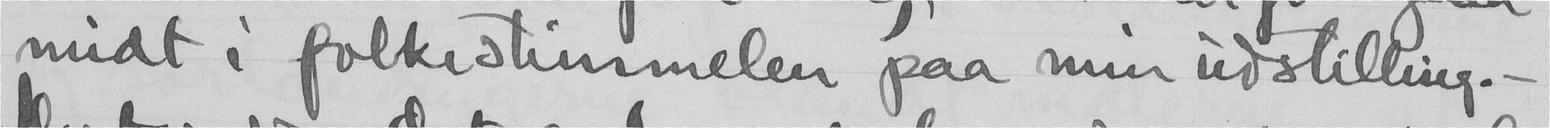midt i folkestimmelen paa min udstilling.
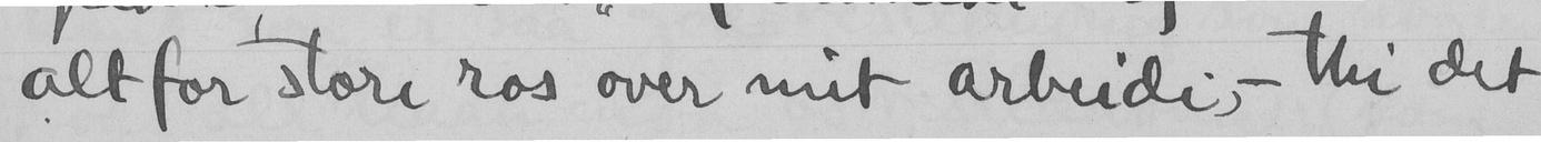alt for store ros over mit arbeide, - thi det
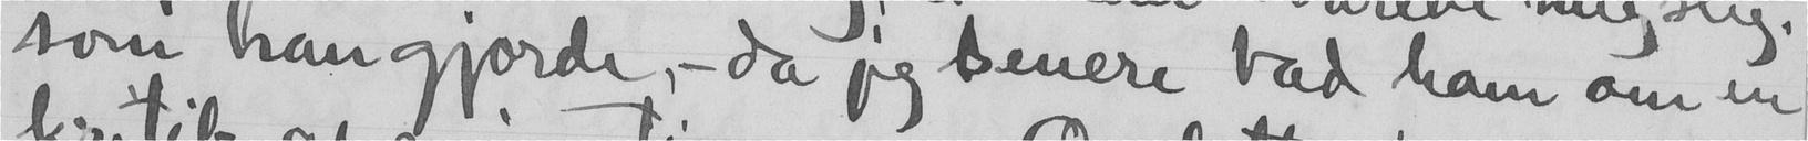som han gjorde, - da jeg senere bad ham om en
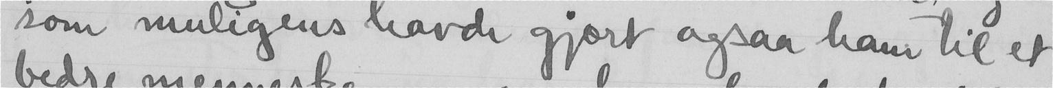som muligens havde gjort ogsaa ham til et
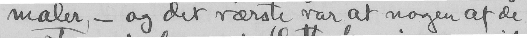maler, - og det værste var at nogen af de
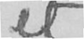et
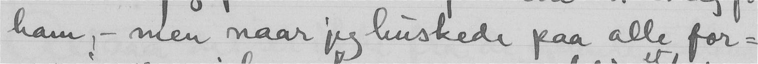ham, - men naar jeg huskede paa alle for-
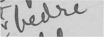bedre
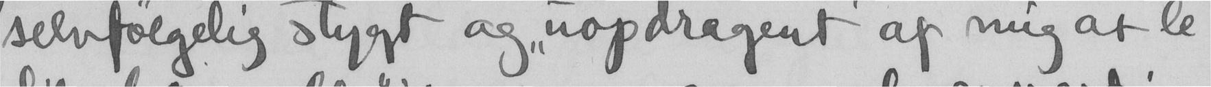selvfølgelig stygt og "uopdragent" af mig at le
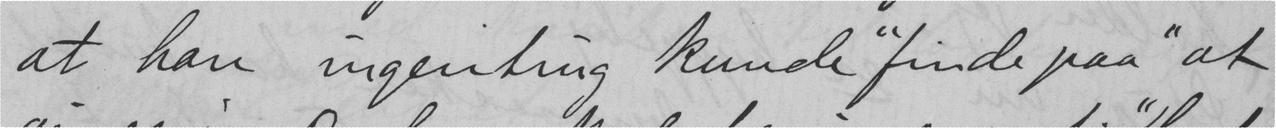at han ingenting kunde finde paa at
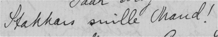Stakkars snille Mand!
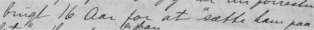brugt 16 Aar for at "sætte ham paa
